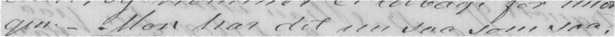gen. - Mor har det nu saa som saa,
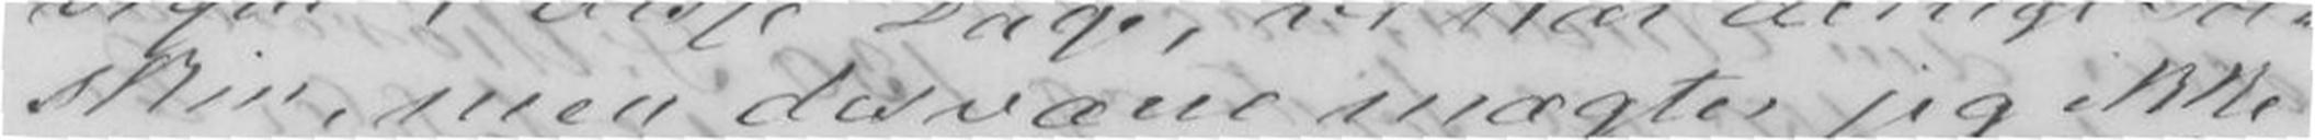skin, men desværre magter jeg ikke
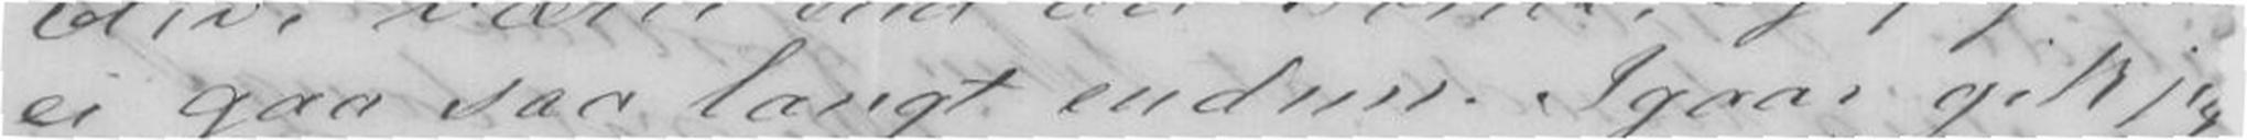ei gaa saa langt endnu. Igaar gik jeg
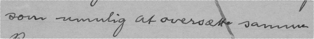som umulig at oversætte samme
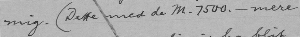mig. (Dette med de M. 7500. - mere
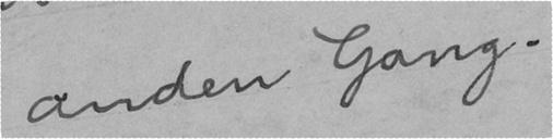anden Gang.
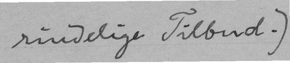rindelige Tilbud.)
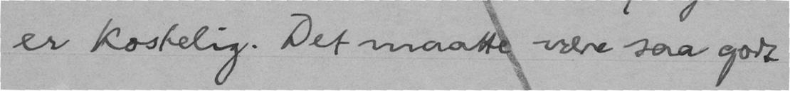er kostelig. Det maatte være saa godt
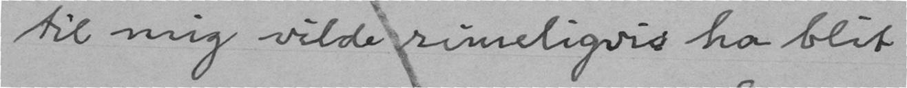til mig vilde rimeligvis ha blit
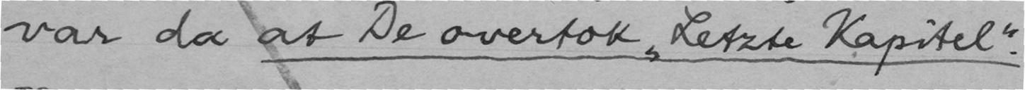var da at De overtok "Letze Kapitel".
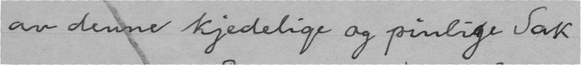av denne kjedelige og pinlige Sak
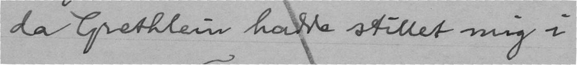da Grethlein hadde stillet mig i
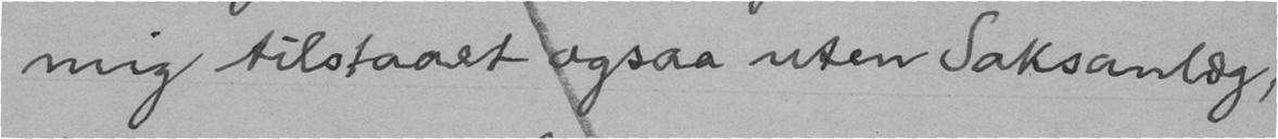mig tilstaaet ogsaa uten Saksanlæg,
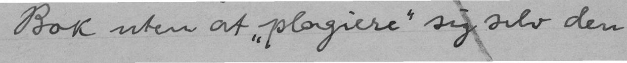Bok uten at "plagiere" sig selv den
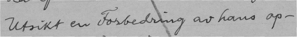Utsikt en Forbedring av hans op-
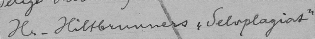Hr. Hiltbrunners "Selvplagiat"
